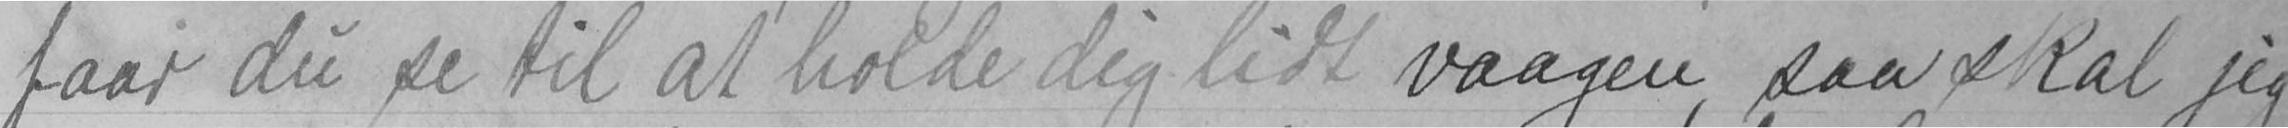faar du se til at holde dig lidt vaagen, saa skal jeg
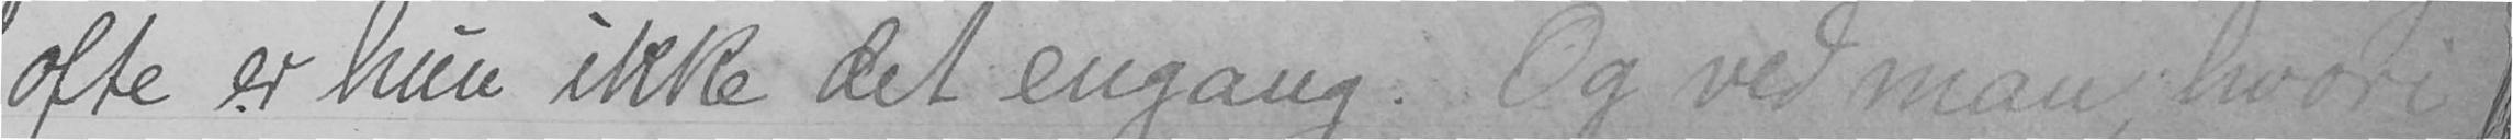ofte er hun ikke det engang. Og ved man, hvori
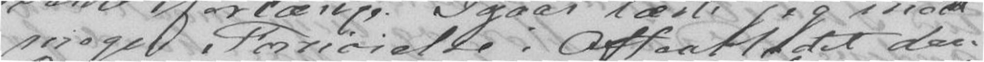meget Fornøielse i Aftenbladet den
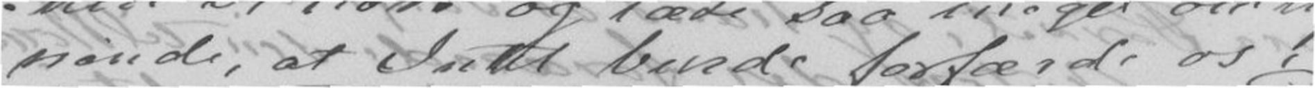nende, at Intet burde forfærde os i
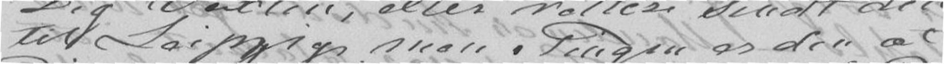til Leipzig, men Tingen er den at
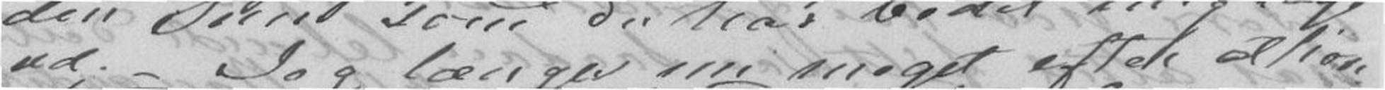ud. - Jeg længes nu meget efter at høre
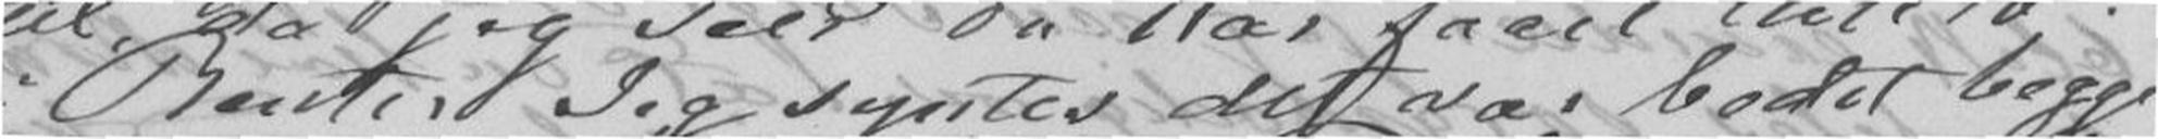i Renter. Jeg syntes det bedst begge
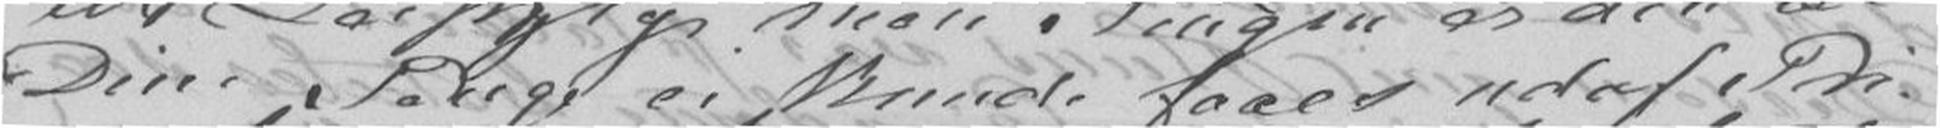Dine Penge ei kunde faaes udaf Pri-
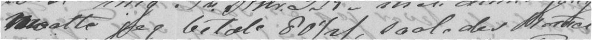maatte jeg betale 80½/, saaledes ko`mer
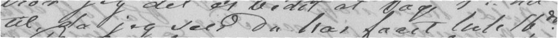til da jef seer Du har faaet hele 16do
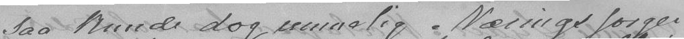saa kunde dog umuelig Naringssorge
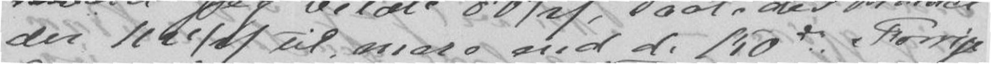der 112½/ til, mere end d. 150do. Forrige
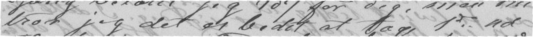tror jeg det er bedst at tage 1do ud
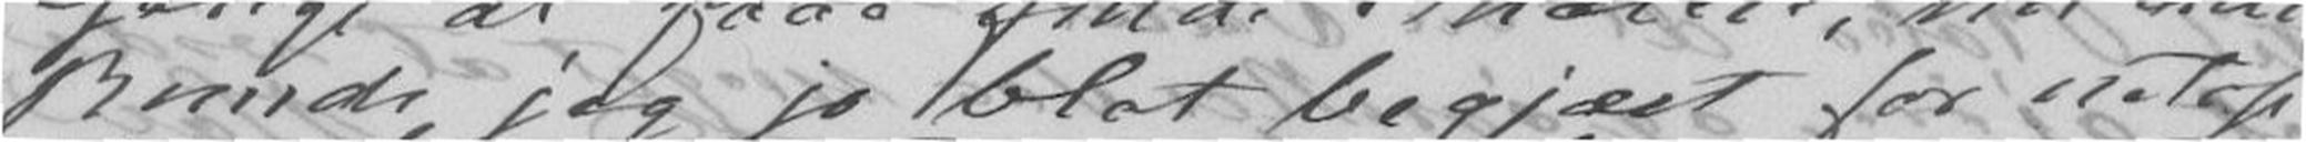kunde jeg jo blot begjært for netop
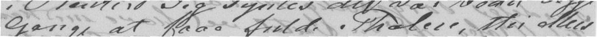Gange at faae fulde Thalere, thi ellers
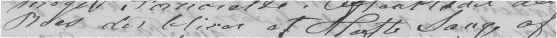Roes der bliver et Hæfte Sange af
